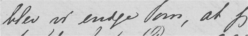blev vi enige om, at jeg
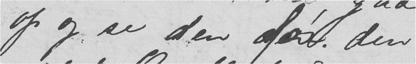op og se den før den
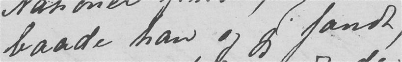baade han og jeg fandt,
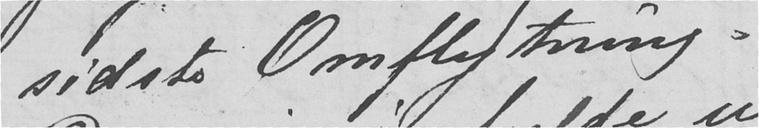sidste Omflytning.
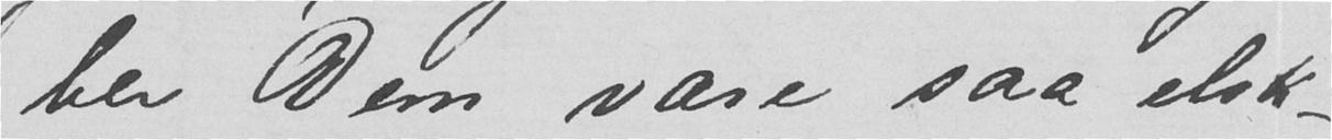ber Dem være saa elsk-
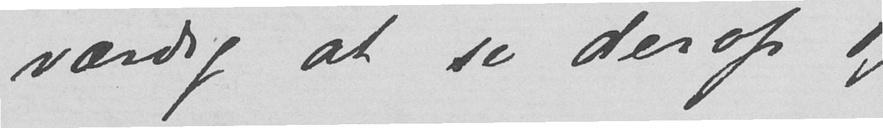værdig at se derop og
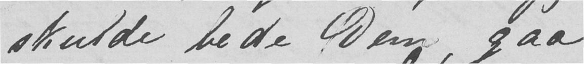skulde bede Dem, gaa
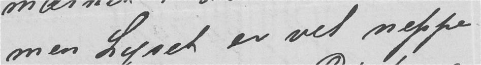men Lyset er vel neppe
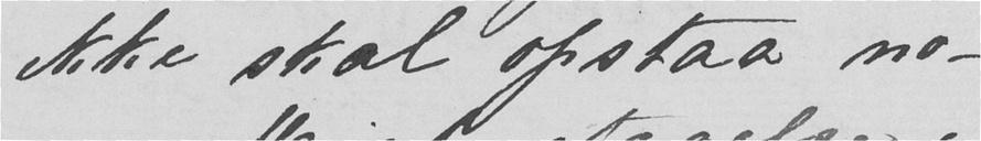ikke skal opstaa no-
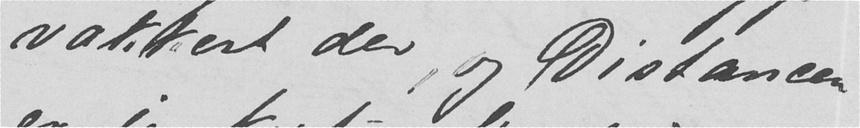vakkert der, og Distancen
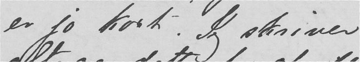er jo kort. Jeg skriver
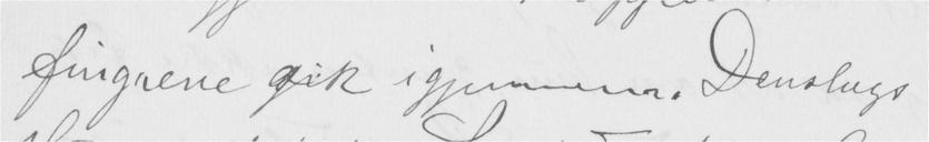fingrene gik igjennem. Denslags
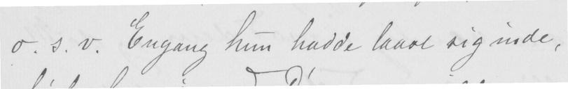o. s. v. Engang hun hadde laast sig inde,
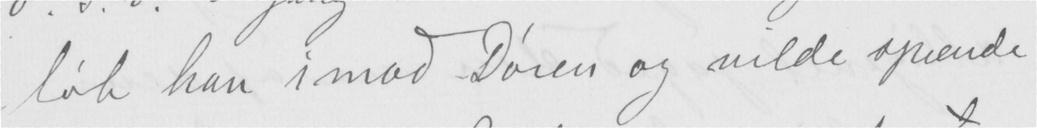løb han imod Døren og vilde spænde
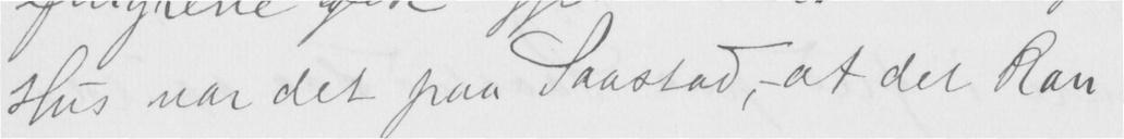Hus var det paa Saastad, – at det kan
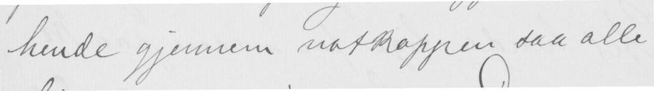hende gjennem natkappen saa alle
